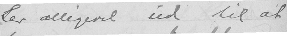Ser alligevel ud til at
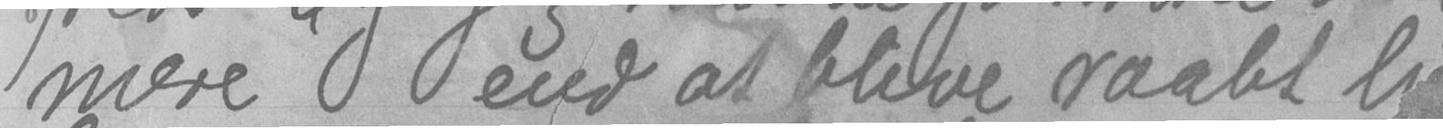mere end at blive raabt h
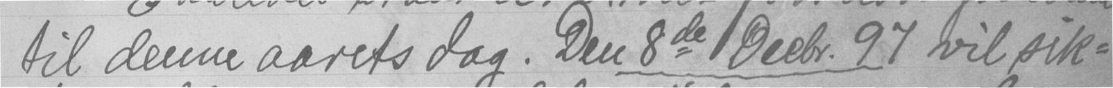til denne aarets dag. Den 8de Decbr. 97 vil sik-
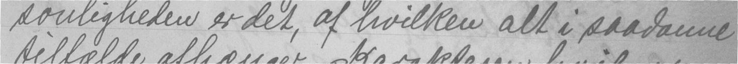sonligheden er det, af hvilken alt i saadanne
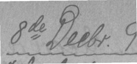8de Decbr. 97.
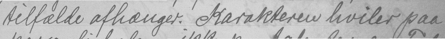tilfælde afhænger. Karakteren hviler paa
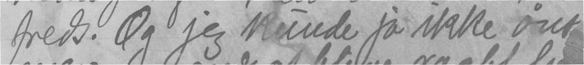freds. Og jeg kunde jo ikke øns
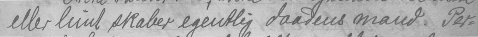eller lunt skaber egentlig daadens mand. Per-
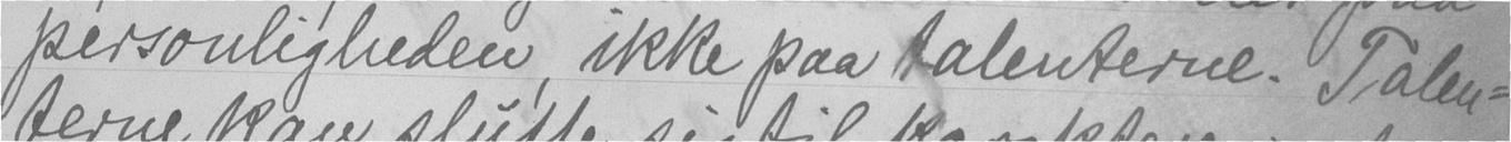personligheden, ikke paa talenterne. Talen-
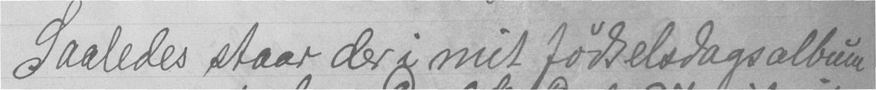Saaledes staar der i mit fødselsdagsalbum
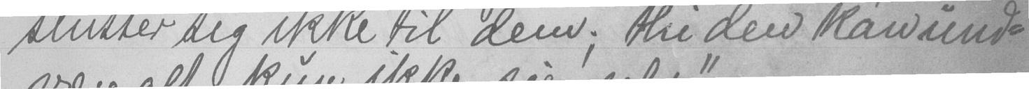slutter sig ikke til dem; ti den kan und-
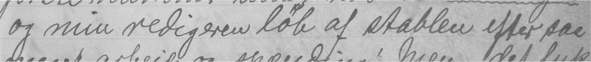og min redigeren løb af stablen efter saa
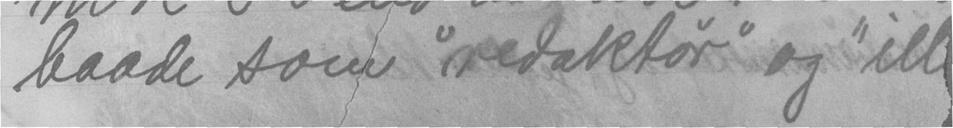baade som "redaktør" og "ill
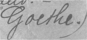(Goethe.)
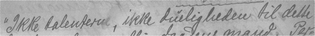"Ikke talenterne, ikke dueligheden til dette
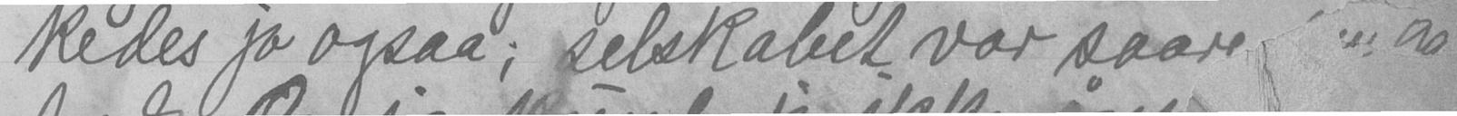kedes jo ogsaa; selskabet var saare
